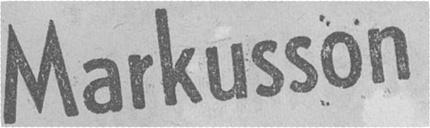Markusson
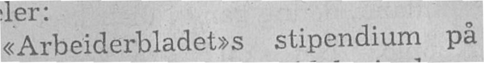"Arbeiderbladet"s stipendium på
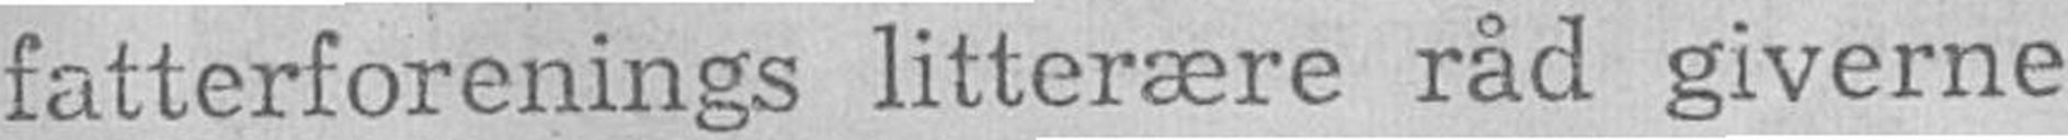fatterforenings litterære råd giverne
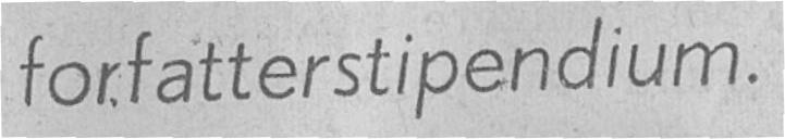forfatterstipendium.
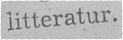litteratur.
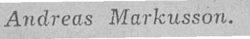Andreas Markusson.
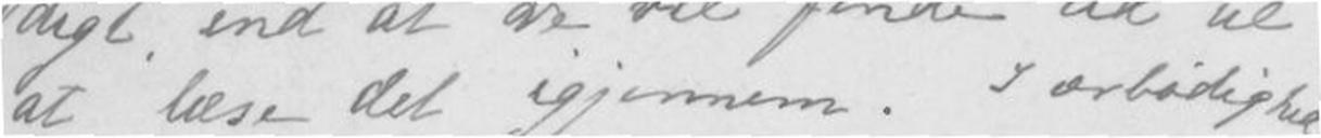at læse det igjennem. I ærbødighed
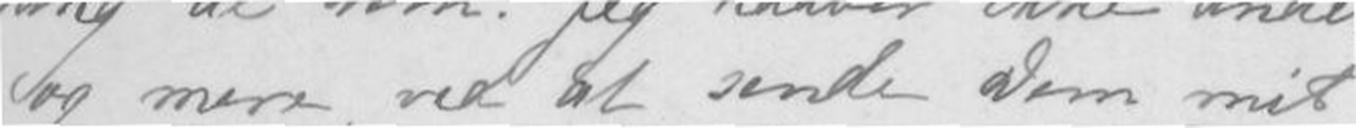og mere ved at sende Dem mit
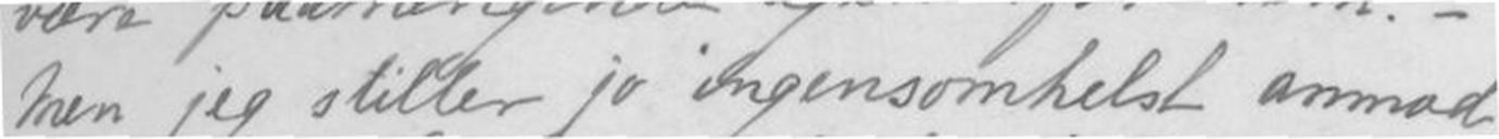Men jeg stiller jo ingensomhelst anmod-
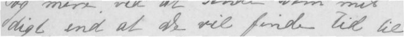digt end at De vil finde tid til
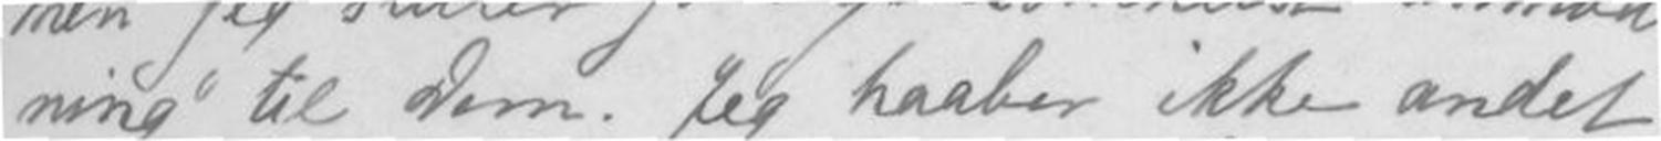ning til Dem. Jeg haaber ikke andet
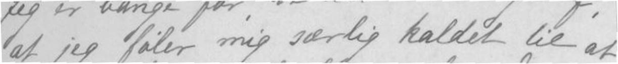at jeg føler mig særlig kaldet til at
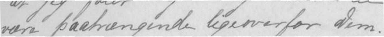være paatrængende ligeoverfor Dem.-
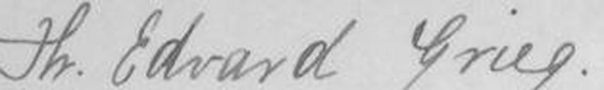Hr. Edvard Grieg!
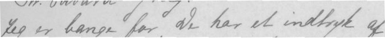Jeg er bange for De har et indtryk af,
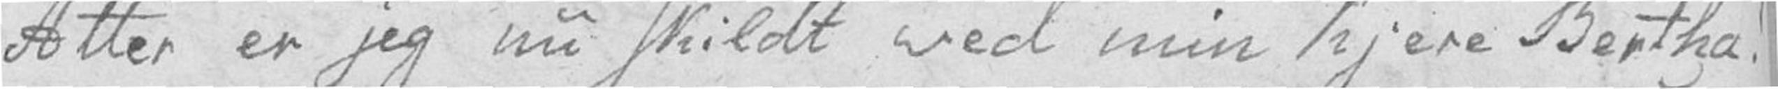Atter er jeg nu skildt ved min kjere Bertha!
